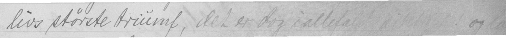livs største triumf, det er dog iallefald sikkert! og lad
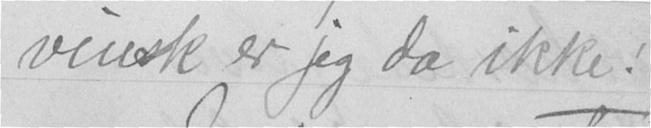vinsk er jeg da ikke!
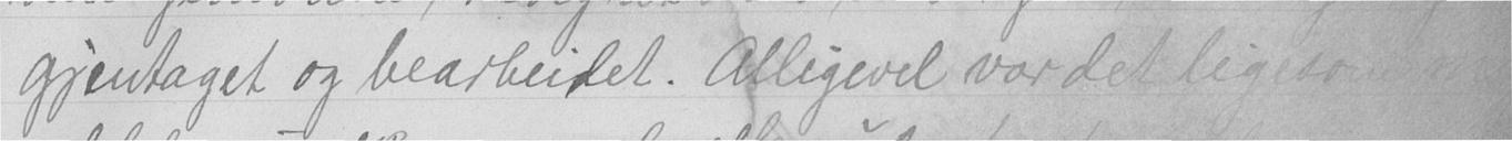gjentaget og bearbeidet. Alligevel var det ligesom
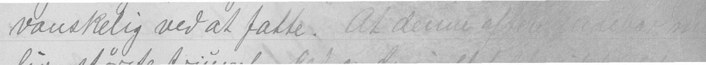vanskelig ved at fatte. At denne aften  mit
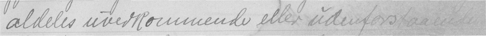aldeles uvedkommende eller udenforstaaende - -
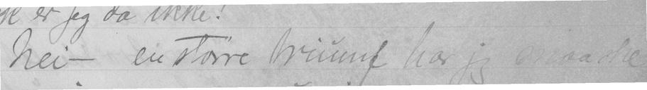Nei - en større triumf har jeg maaske
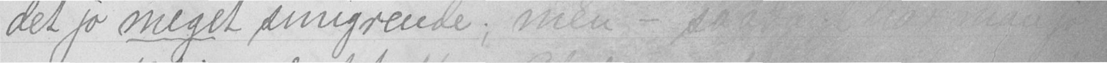det jo meget smigrende; men - saadant har man jo
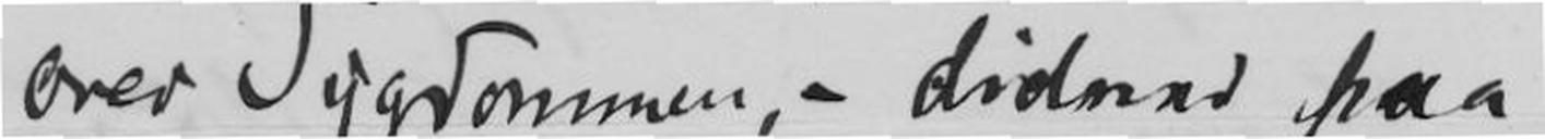over Sygdommen, - didned paa
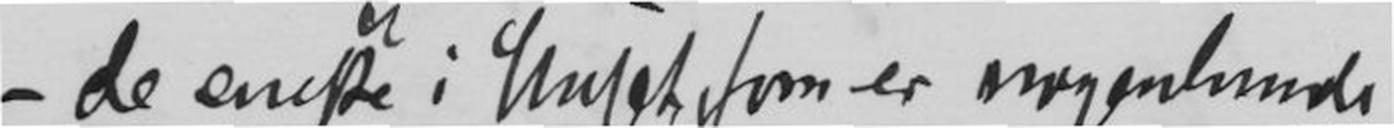-de eneste i huset som er nogenlunde
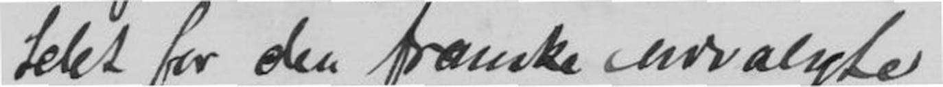telet for den franske udvalgte
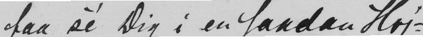faa se Dig i en saadan Høj-
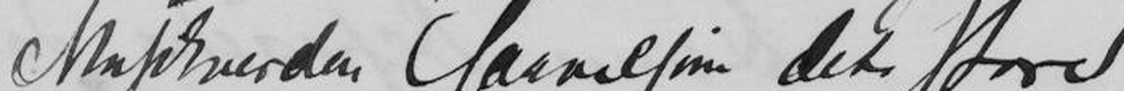Musikverden Saavelsom dets store
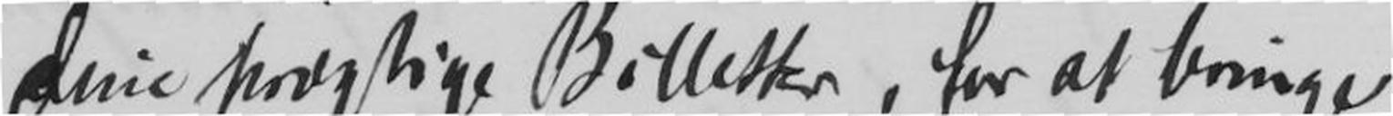dine prægtige Billetter, for at bringe
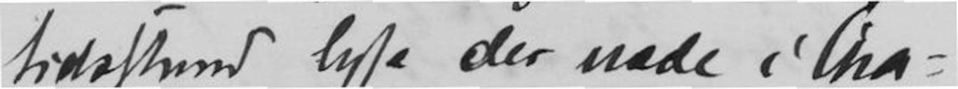tidsstund lyse der nede i Cha-
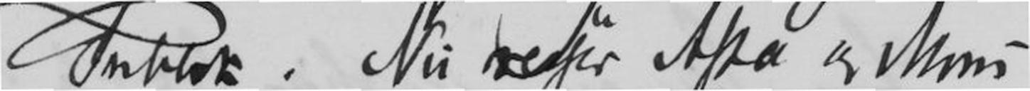Publik. Nu rejer Asta og Mons
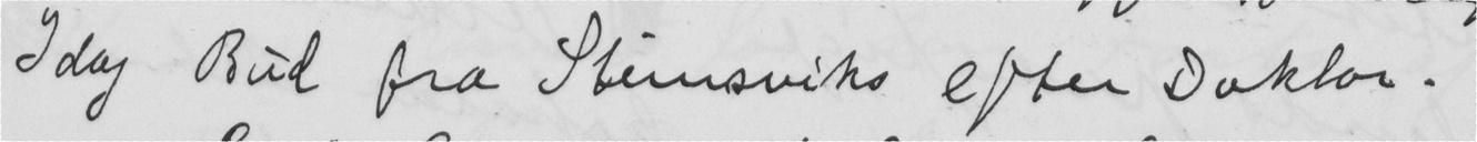Idag Bud fra Steinsviks efter Doktor.
